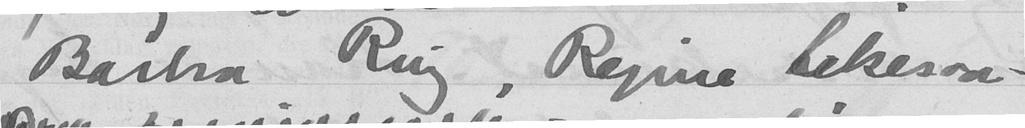Barbra Ring, Regine likesaa -
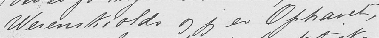Werenskiolds og jeg er Ophavet,
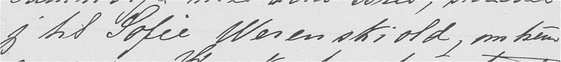jeg til Sofie Werenskiold, om hun
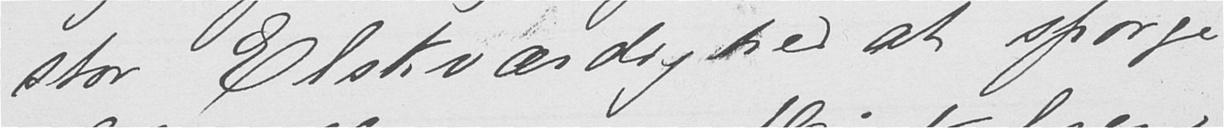stor Elskværdighed at spørge
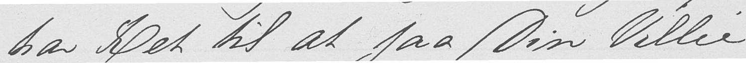har Ret til at faa Din Villie
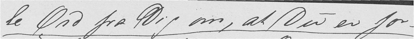le Ord fra Dig om, at Du er for-
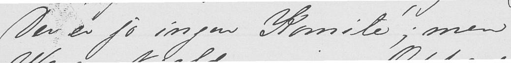Der er jo ingen Komite; men
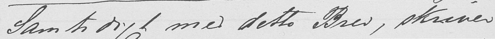Samtidigt med dette Brev, skriver
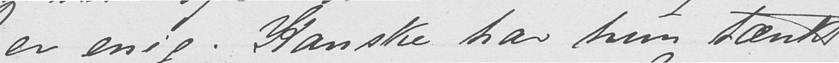er enig. Kanske har hun tænke
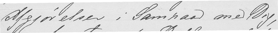Afgjørelser i Samraad med Dig,
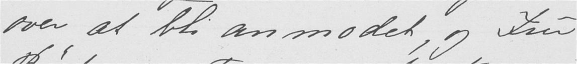over at bli anmodet, og Fru
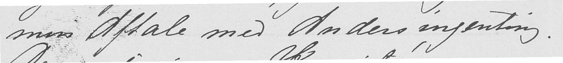min Aftale med Anders ingenting.
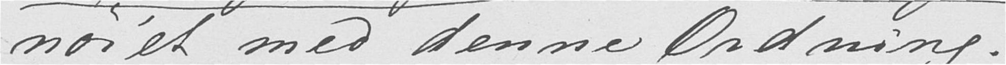nøiet med denne Ordning.
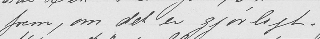frem, om det er gjørligt.
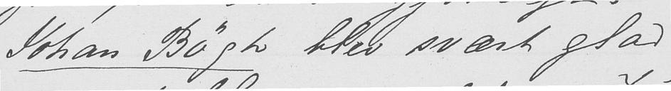Johan Bøgh blev svært glad
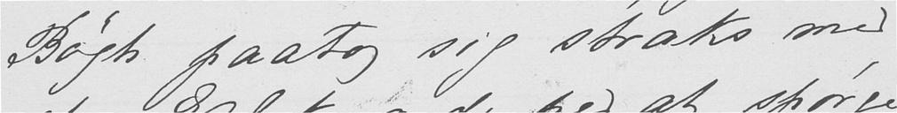Bøgh paatog sig straks med
